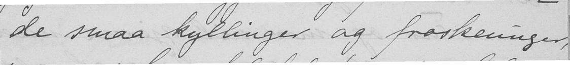de smaa kyllinger og froskeunger
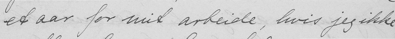et aar for mit arbeide, hvis jeg ikke
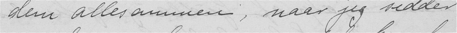dem allesammen, naar jeg sidder
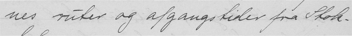nes ruter og afgangstider fra Stok-
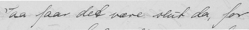Saa faar det være slut da, for
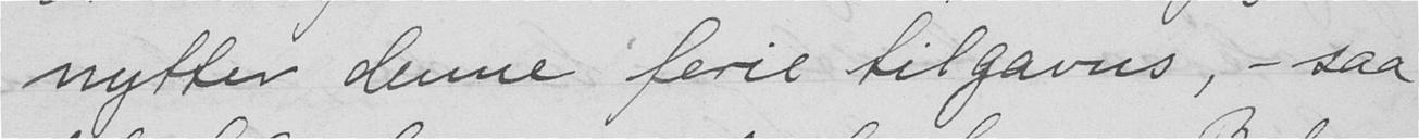nytter denne ferie tilgavns, - saa
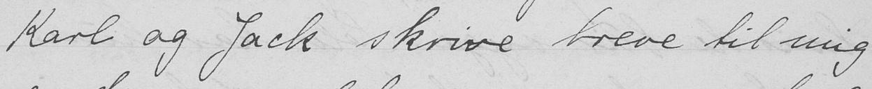Karl og Jack skrive breve til mig
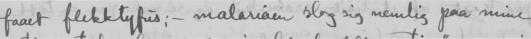faaet flekktyfus - malariaen slog sig nemlig paa mine
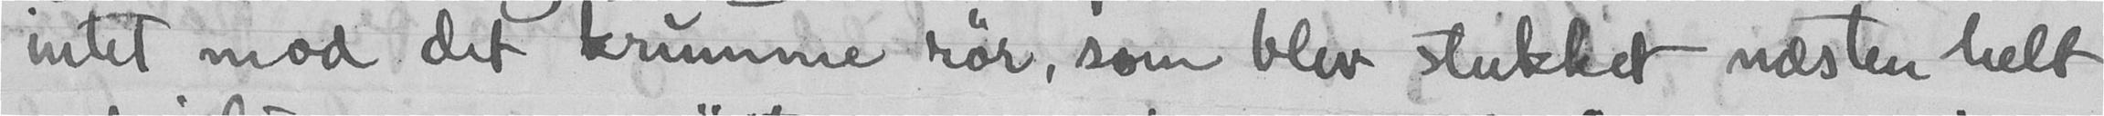intet mod det krumme rør, som bliv stukket næsten helt
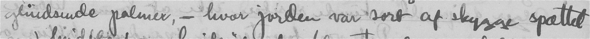glindsende palmer, - hvor jorden var sort af skygge spættet
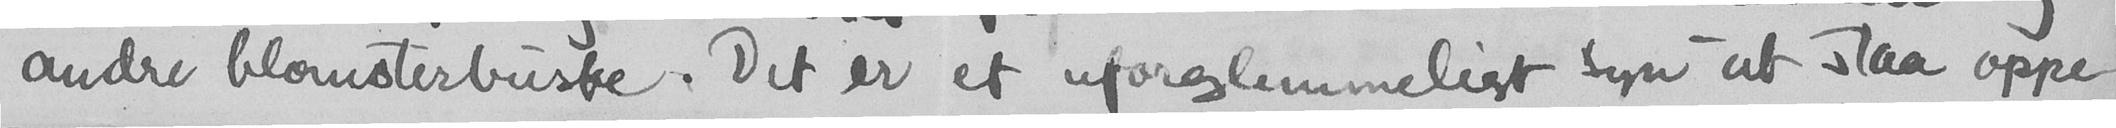andre blomsterbuske. Det er et uforglemmeligt syn at staa oppe
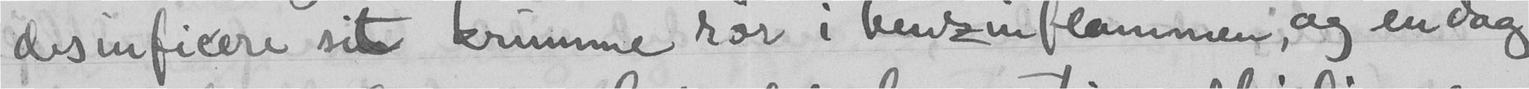disinficere sit krumme ror i benzinflammen, og en dag
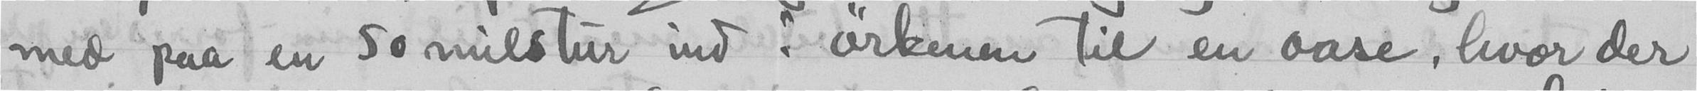med paa en 50 milstur ind i ørkenen til en oase, hvor der
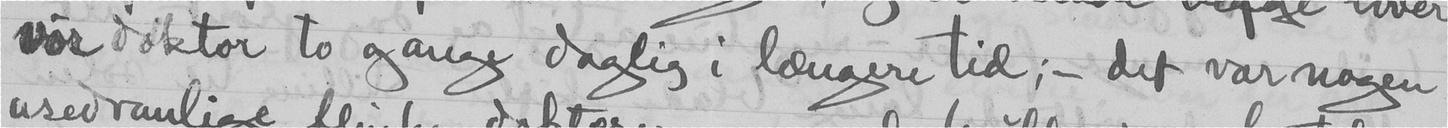vor doktor to gange daglig i længere tid ; - det var nogen
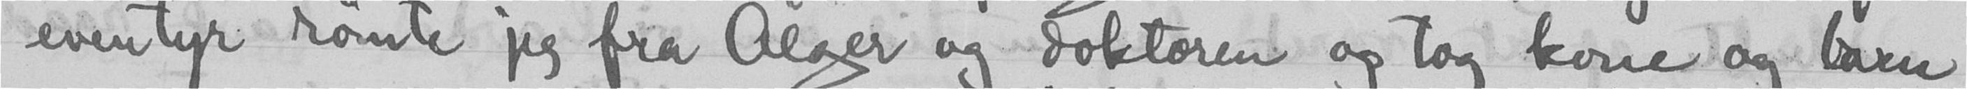eventyr rømte jeg fra Alger og doktoren og tog kone og barn
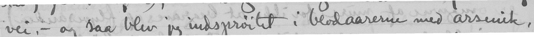vei, - og saa blev jeg indsprøitet i blodaarerne med arsenik,
